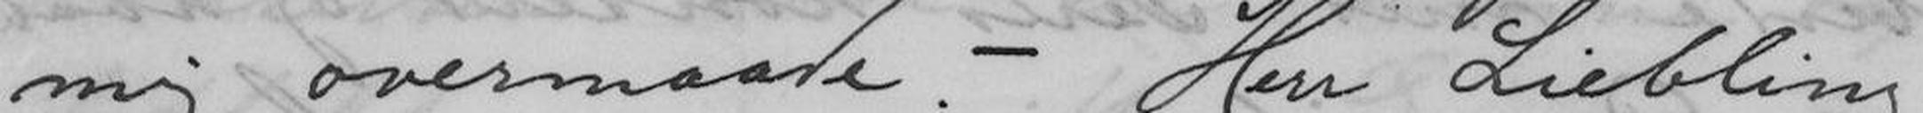mig overmaade. - Herr Liebling
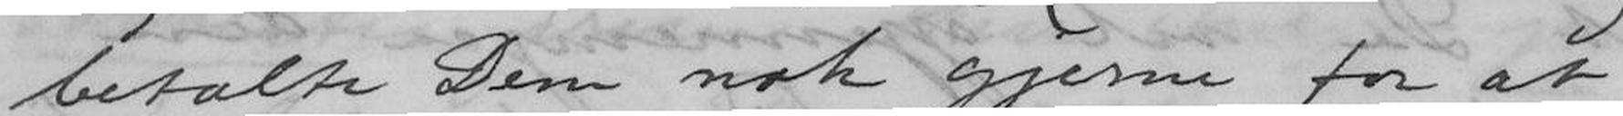betalte Dem nok gjerne for at
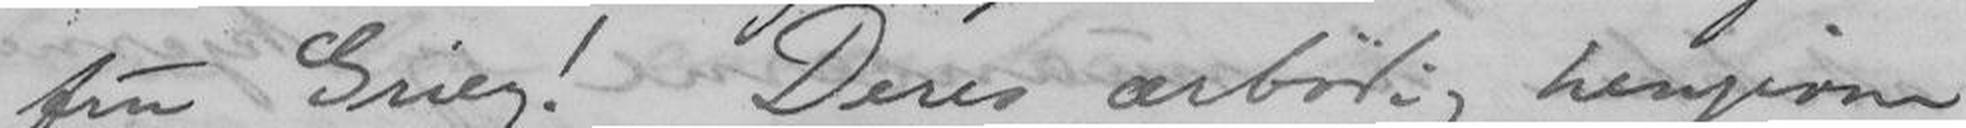Fru Grieg! Deres ærbødig hengivne
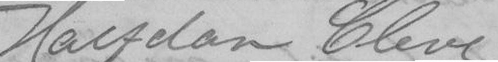Halfdan Cleve.
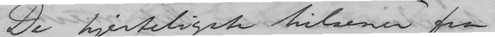De hjerteligste hilsener fra
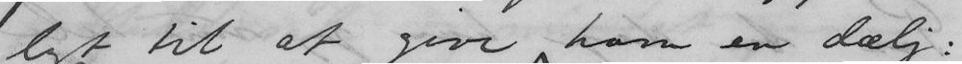lyst til at give ham en dælj:
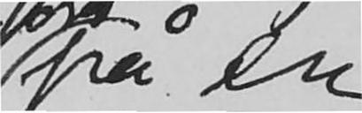på en
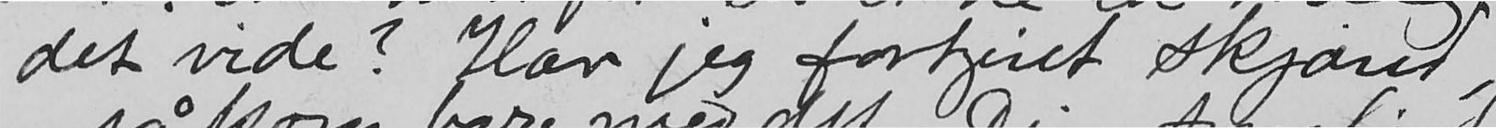det vide? Har jeg fortjent skjænd,
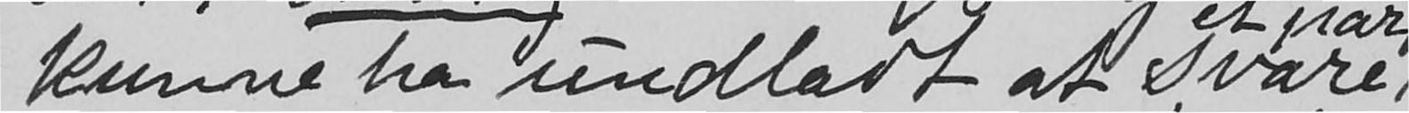kunne ha undladt at svare
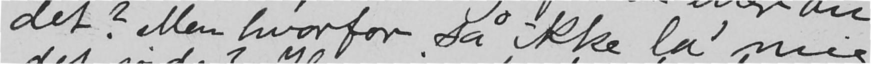det? Men hvorfor så ikke la mig
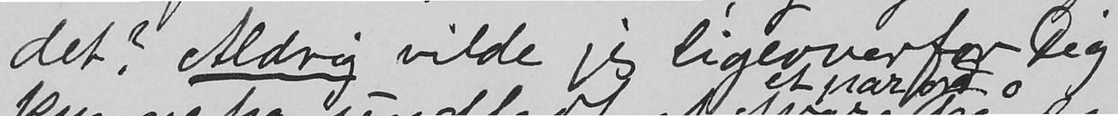det? Aldrig vilde jeg ligeoverfor Dig
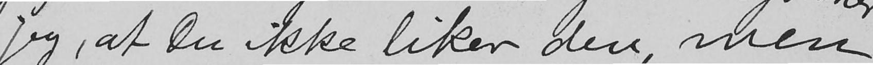Jeg, at Du ikke liker den, men
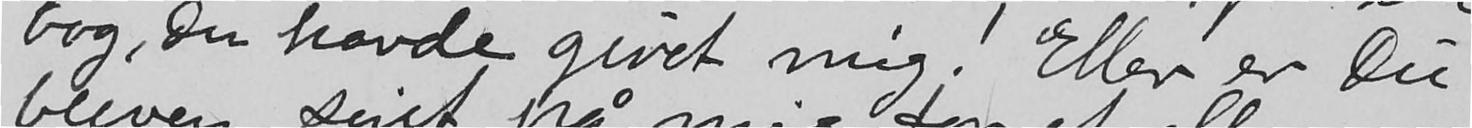bog, Du havde givet mig! Eller er Du
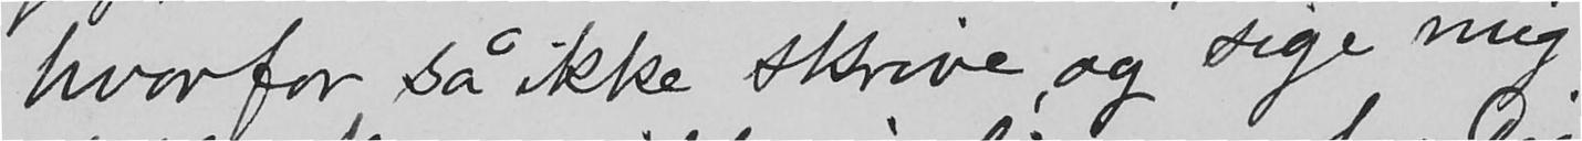hvorfor så ikke skrive, og sige mig
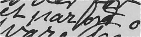et par ord
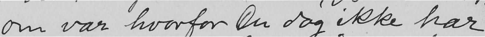om var hvorfor Du dog ikke har
